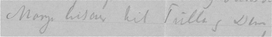Mange hilsener til Tulla og Dem
